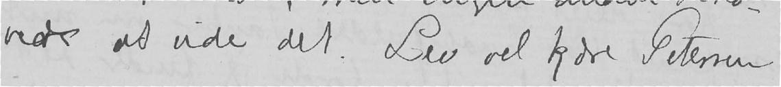vede at vide det. Lev vel kjære Petersen
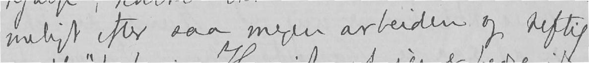meligt efter saa megen arbeiden og heftig
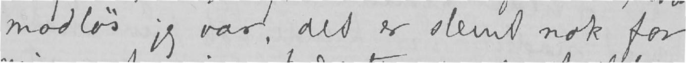modløs jeg var, det er slemt nok for
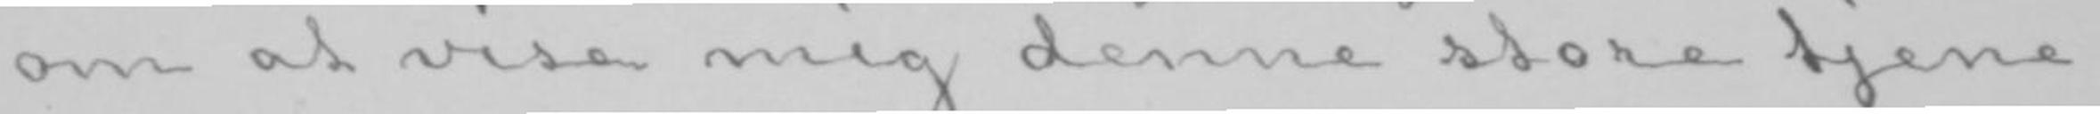om at vise mig denne store tjene-
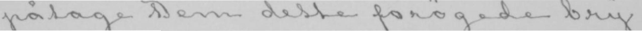påtage Dem dette forøgede bry-
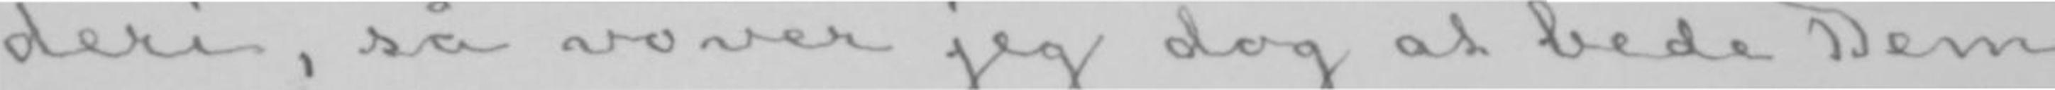deri, så vover jeg dog at bede Dem
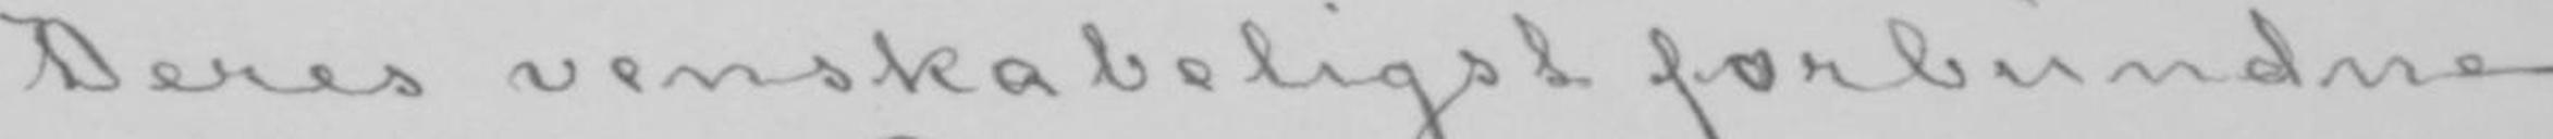Deres venskabeligst forbundne
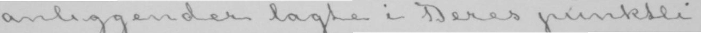anliggender lagte i Deres punktli-
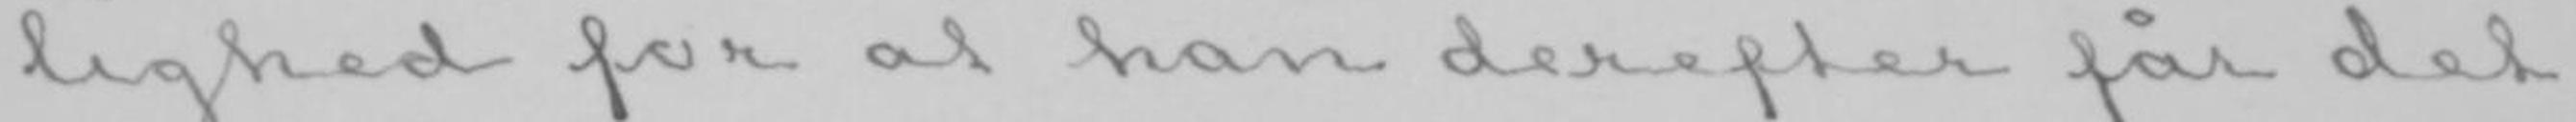lighed for at han derefter får det
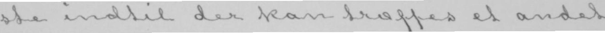ste indtil der kan træffes et andet
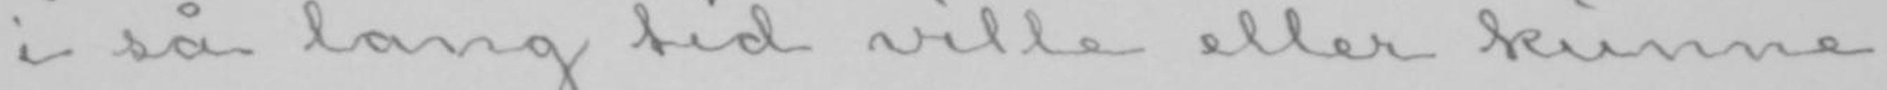i så lang tid ville eller kunne
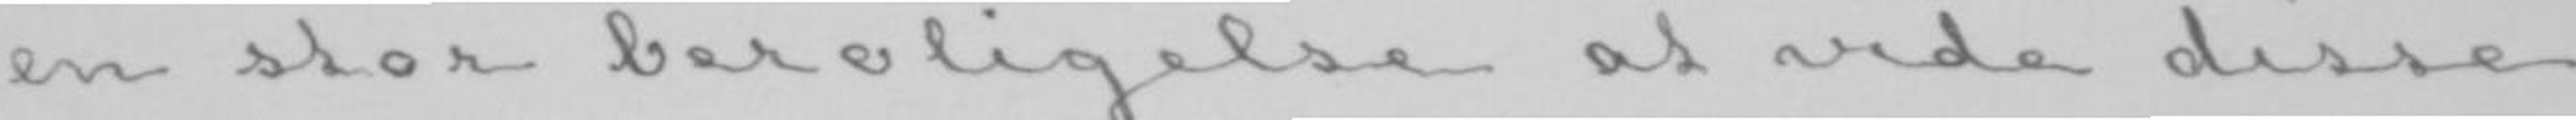en stor beroligelse at vide disse
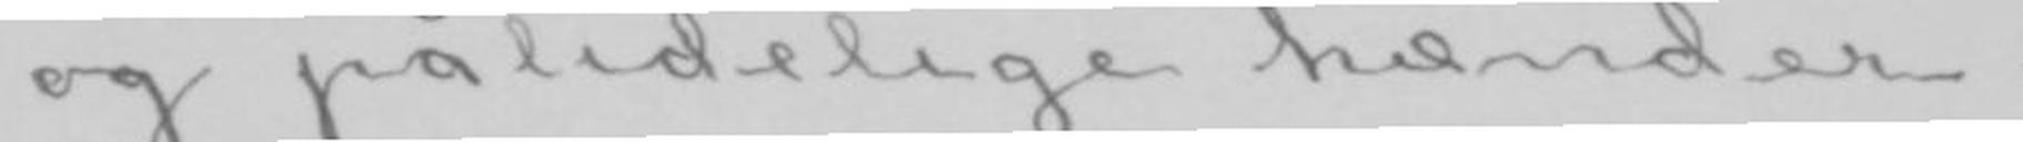ge og pålidelige hænder.
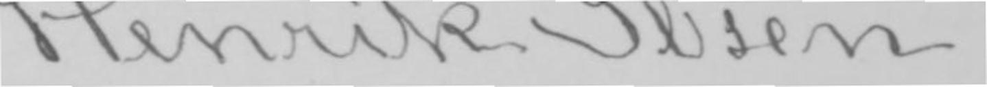Henrik Ibsen.
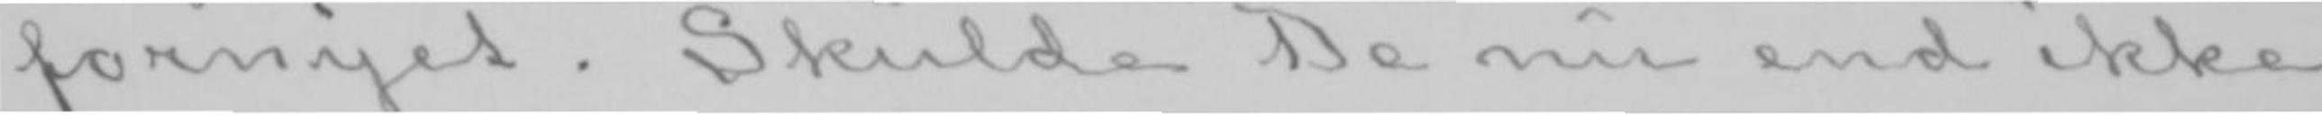fornyet. Skulde De nu end ikke
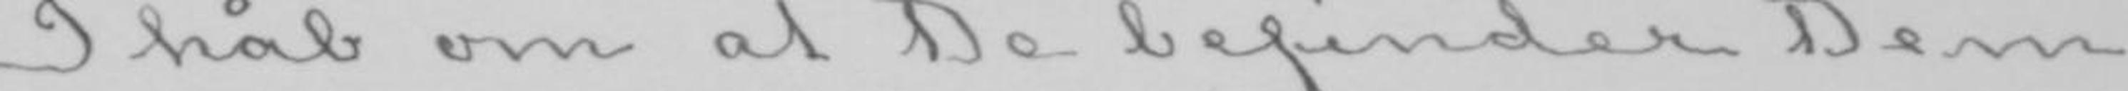I håb om at De befinder Dem
